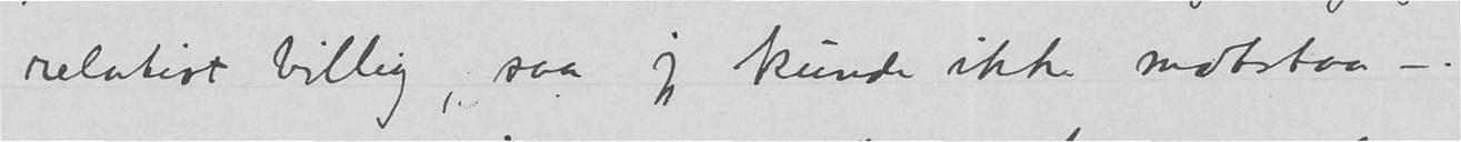relativt billig, saa jeg kunde ikke motstaa –.
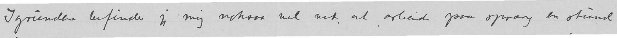Igrunden befinder jeg mig noksaa vel ved, at arbeide paa spræng en stund
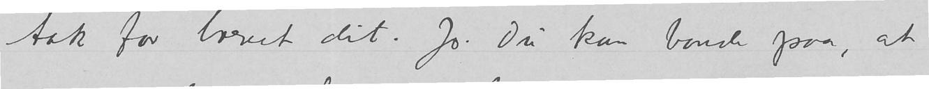tak for brevet dit. Jo. Du kan bande paa, at
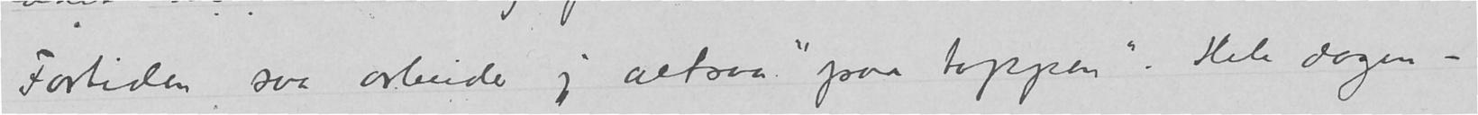Fortiden saa arbeider jeg altsaa «paa toppen». Hele dagen –
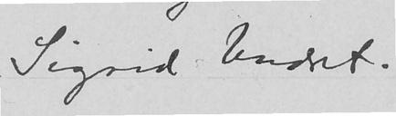Sigrid Undset
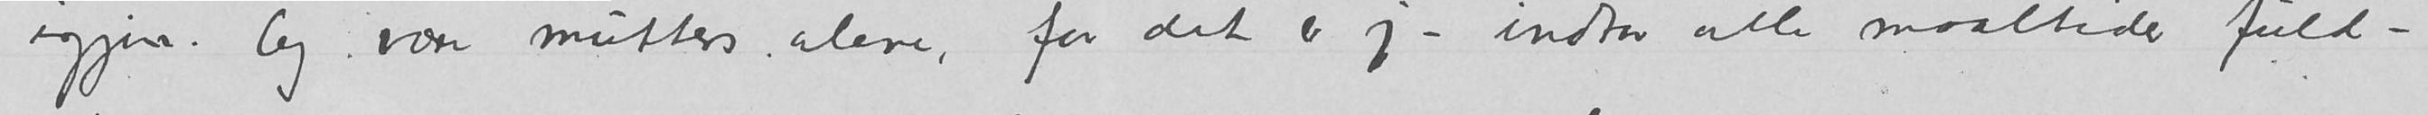igjen. Og som mutters alene, for det er jeg – indtar alle maaltider fuld –
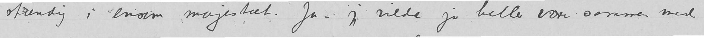stændig i ensom majestet. Ja, jeg vilde jo heller være sammen med
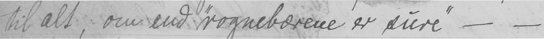til alt, om end "rognebærene er sure" - -
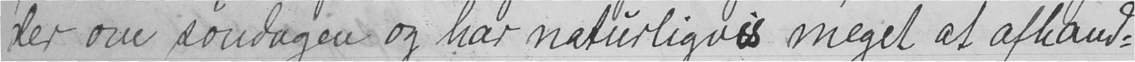der om søndagen og har naturligvis meget at afhand-
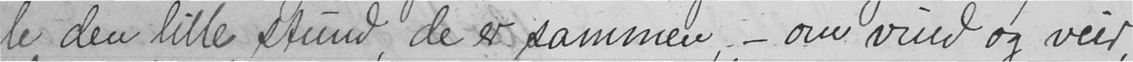le den lille stund, de er sammen, - om vind og veir,
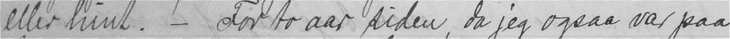eller hint. - For to aar siden, da jeg ogsaa var paa
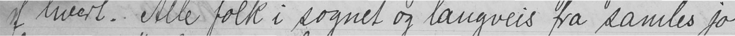af hvert. Alle folk i sognet og langveis fra samles jo
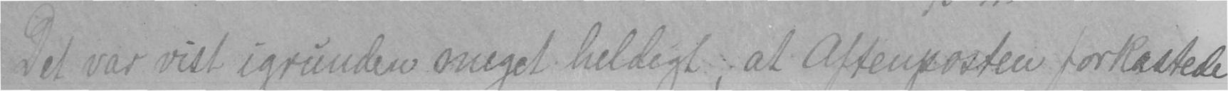Det var vist igrunden meget heldigt, at Aftenposten forkastede
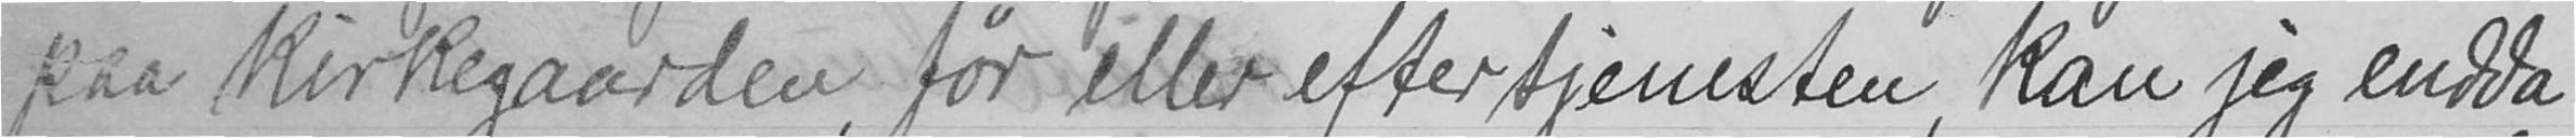paa kirkegaarden, før eller efter tjenesten, kan jeg endda
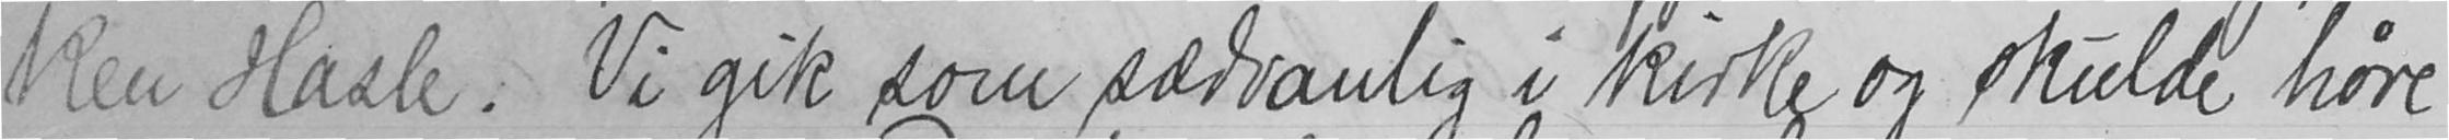ken Hasle. Vi gik som sædvanlig i kirke og skulde høre
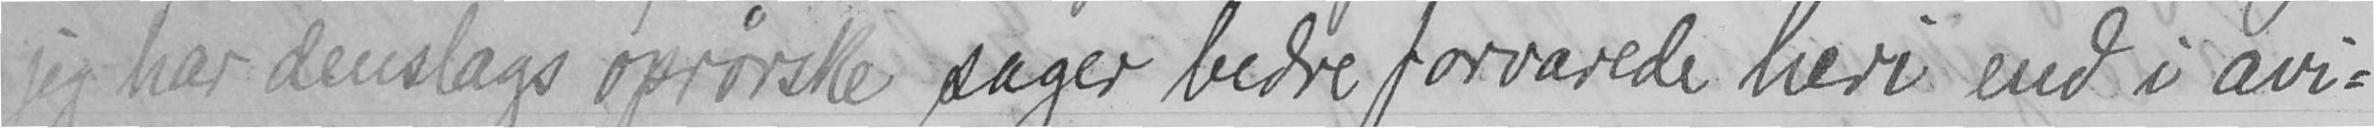jeg har denslags oprørske sager bedre forvarede heri end i avi-
